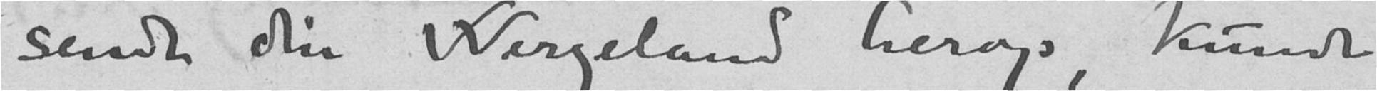sende din Wergeland herop, kunde
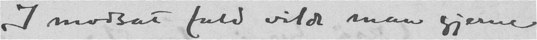I modsat fald vilde man gjerne
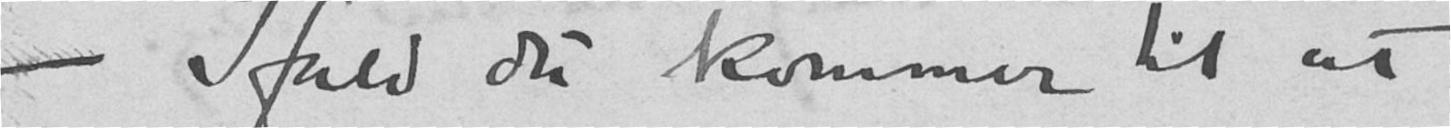- Ifald du kommer til at
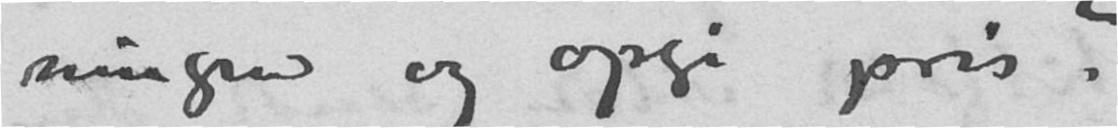ningen og opgi pris?
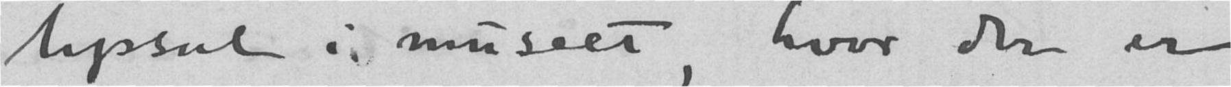lyssal i museet, hvor der er
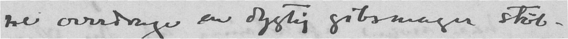ne overdrage en dygtig gipsmager støb-
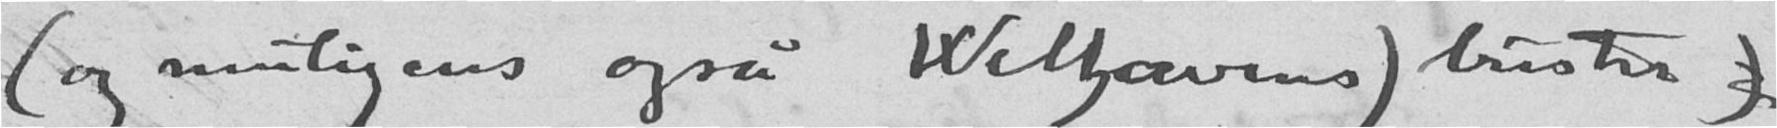(og muligens også Welhavens) buster )
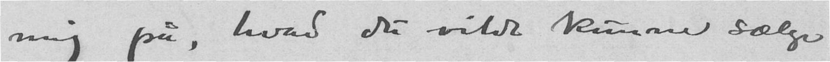mig på, hvad du vilde kunne sælge
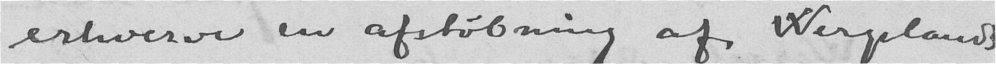erhverve en afstøbning af Wergelands
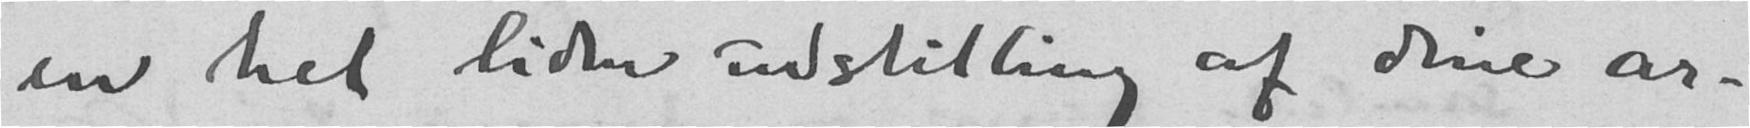en hel liden udstilling af dine ar-
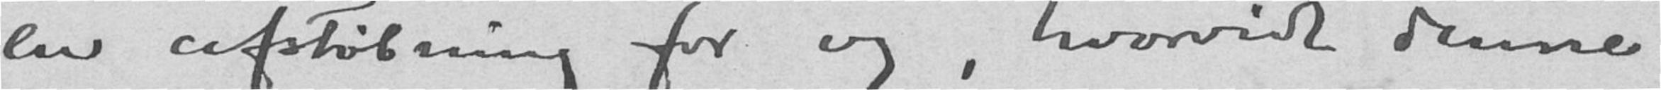en afstøbning for og, hvorvidt denne
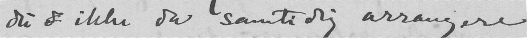du i ikke da samtidig arrangere
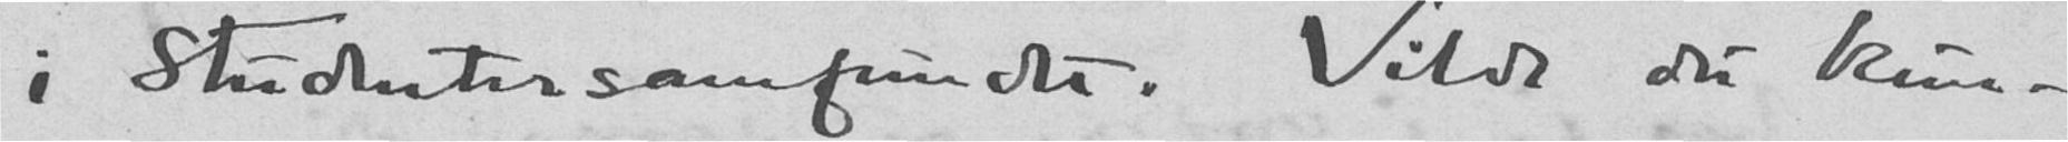i Studentersamfundet. Vilde du kun-
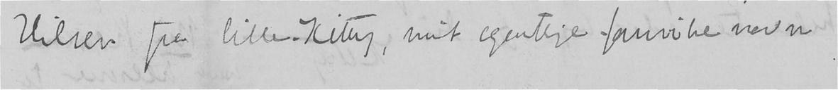Hilsen fra lille-Kitty, mit egentlige familienavn.
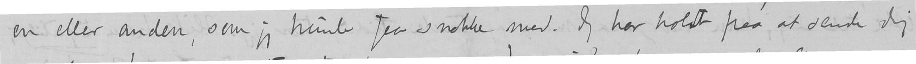en eller anden, som jeg kunde faa snakke med. Jeg har holdt paa at sende dig
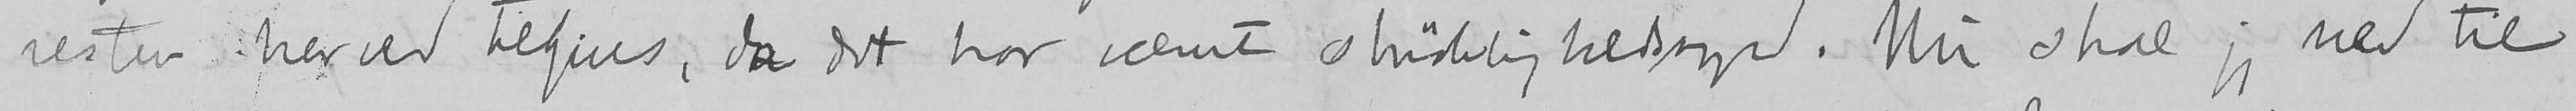resten herved tilgives, da det har været skrøbelighedssynd. Nu skal jeg ned til
